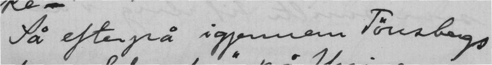Så efterpå igjennem Tønsbergs
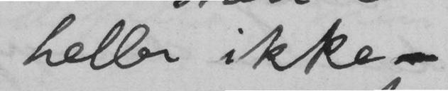heller ikke -
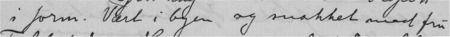i form. Vært i byen og snakket med fru
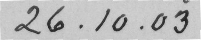26.10.03
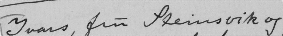Ivars, fru Steinsvik og
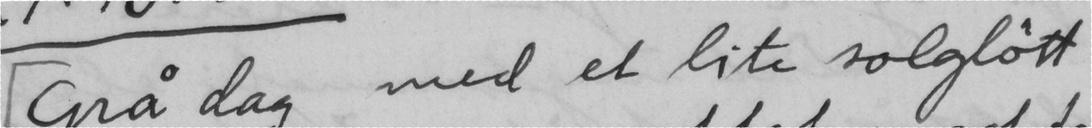Grå dag med et lite solgløtt
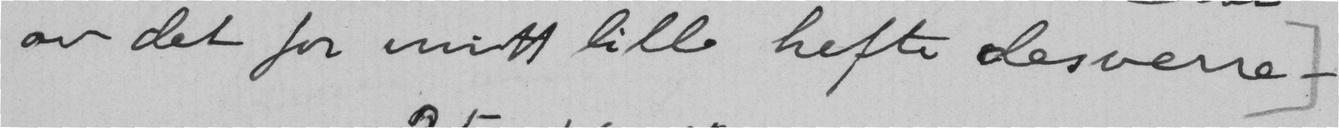av det for mitt lille hefte desverre-
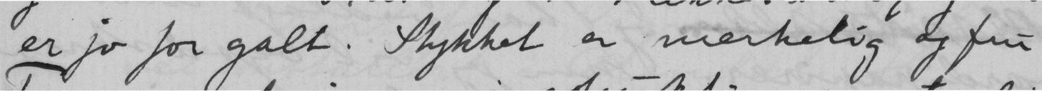er jo for galt. Stykket er merkelig og fru
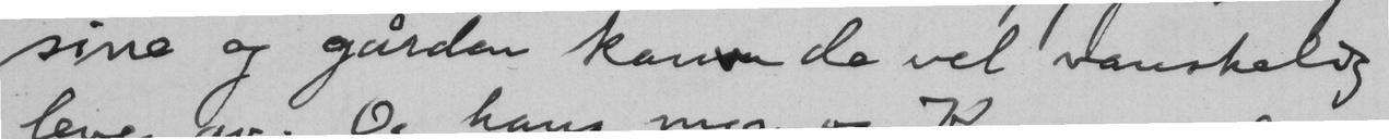sine og gården kann de vel vanskelig
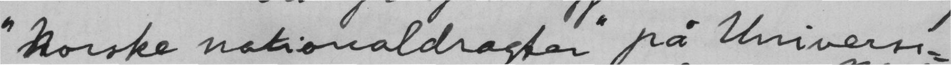"norske nationaldragter" på Universi-
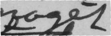noget
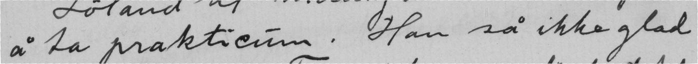å ta prakticum. Han så ikke glad
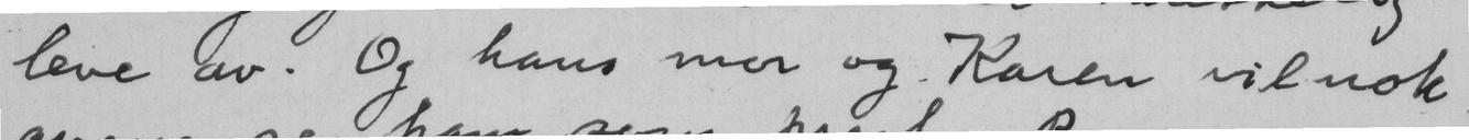leve av. Og hans mor og Karen vil nok
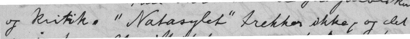og kritik. "Natasylet" trekker ikke, og det
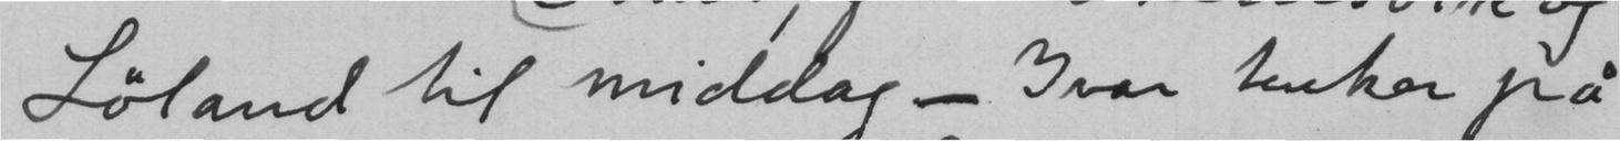Løland til middag – Ivar tenker på
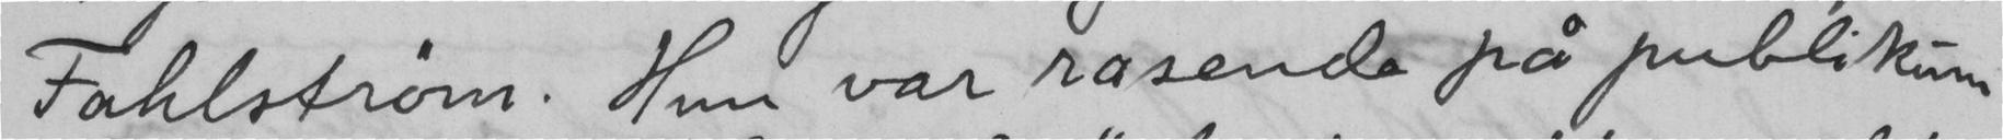Fahlstrøm. Hun var rasende på publikum
